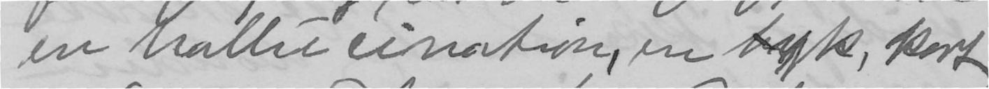en hallucination, en tyk, kort
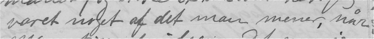været noget af det man mener, når
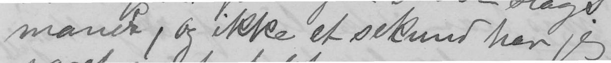manér, og ikke et sekund har jeg
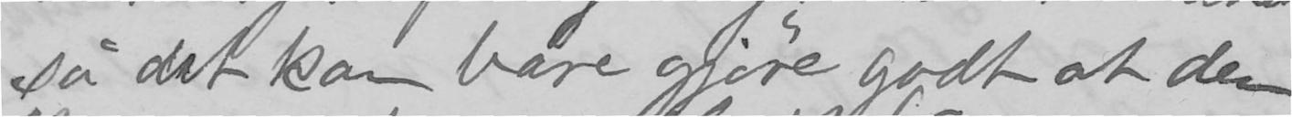så det kan bare gjøre godt at den
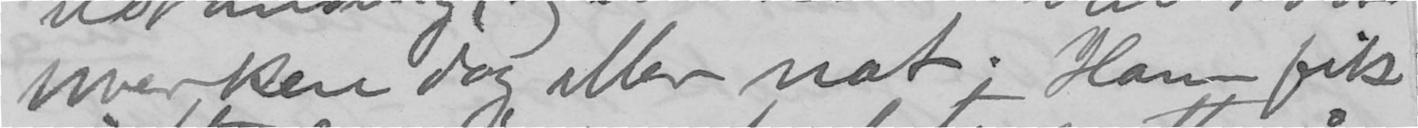hverken dag eller nat; Han fik
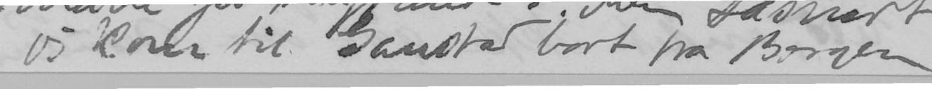jeg kom til Gaustad bort fra Bergen
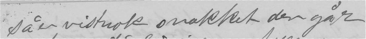så er vistnok snakket der går
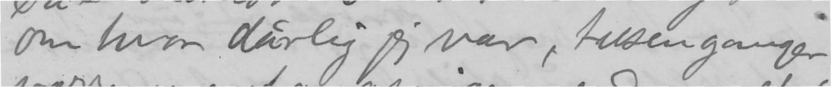om hvor dårlig jeg var, tusen ganger
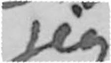jeg
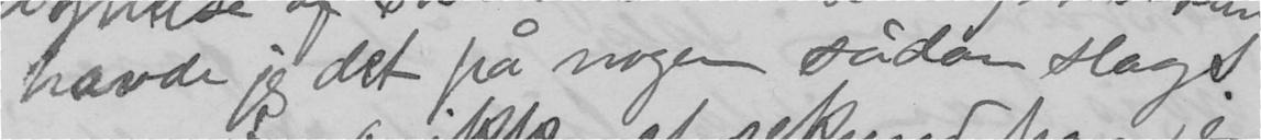havde jeg det på nogen såden slags
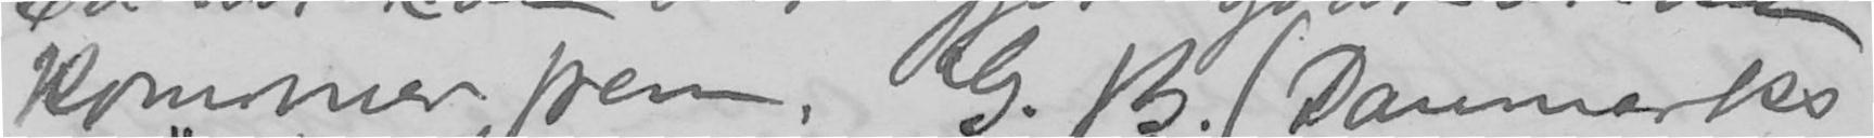kommer frem. G.B.  (Danmarks
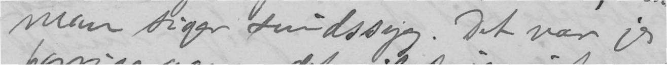man siger sindsyg. Det var jeg
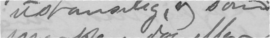ustanselig, og som
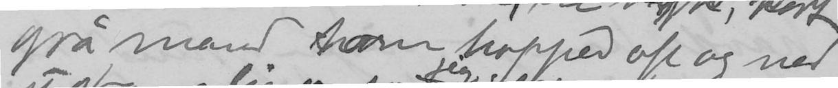grå mand som hopped op og ned
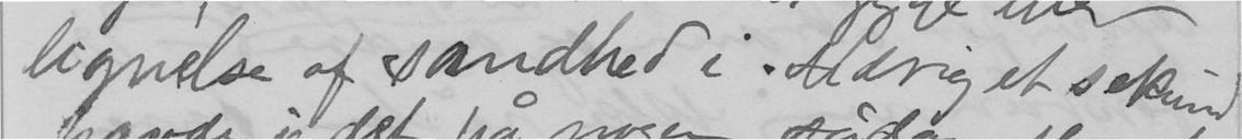lignelse af sandhed i. Aldrig et sekund
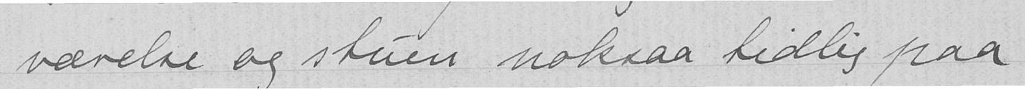værelse og stuen noksaa tidlig paa
